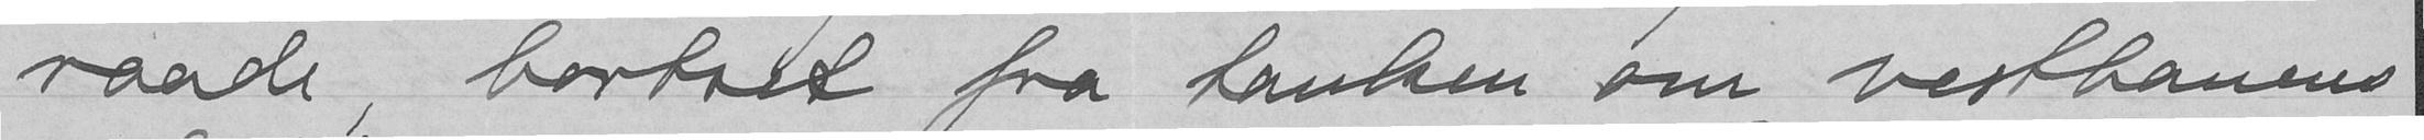raade, bortset fra tanken om vestbanens
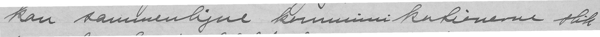kan sammenligne kommunikationerne slik
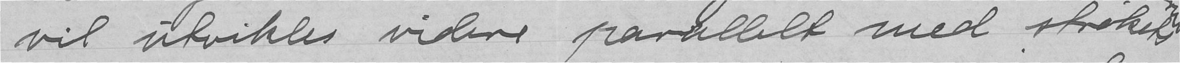vil utvikles videre parallelt med strøkets
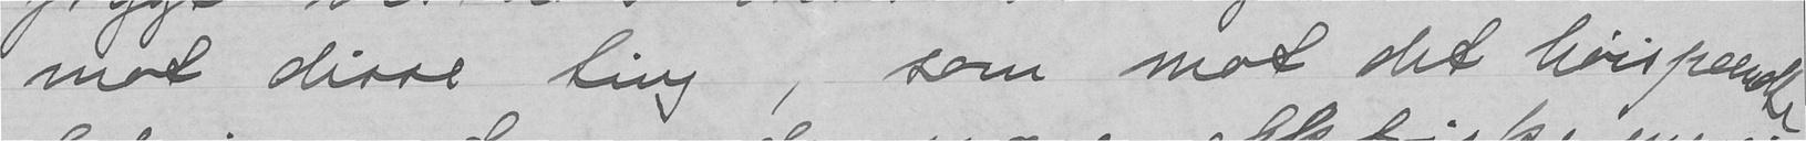mot disse ting, som mot det høispendte
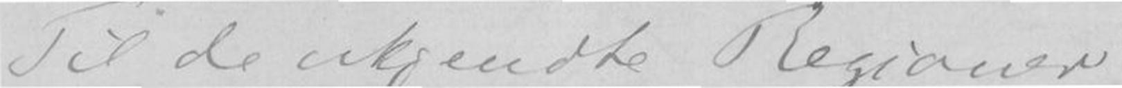Til de ukjendte Regioner
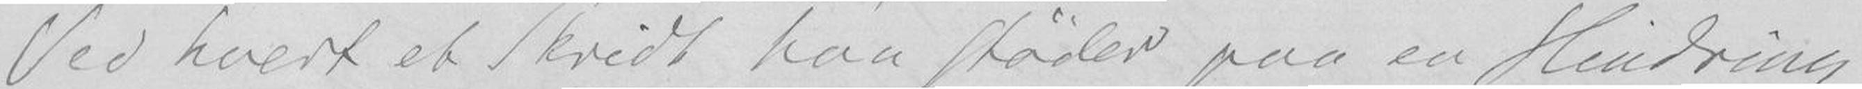Ved hvert et Skridt han støder paa en Hindring
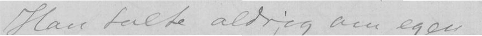Han talte aldrig om egen
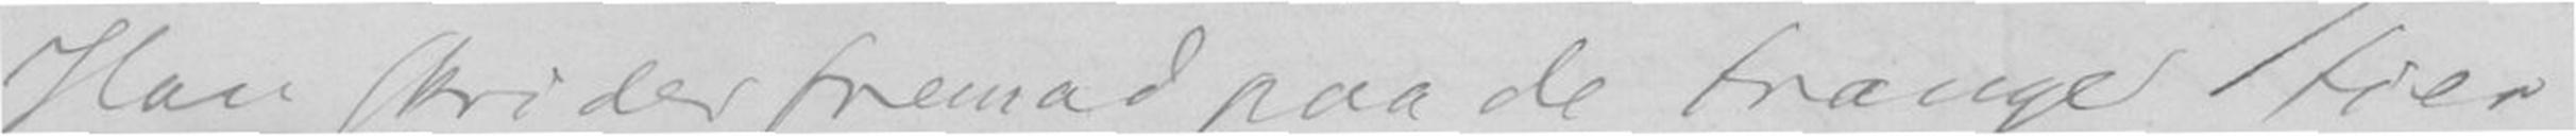han skrider fremad paa de trange Stier
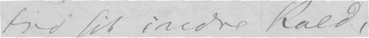fra sit indre Kald,
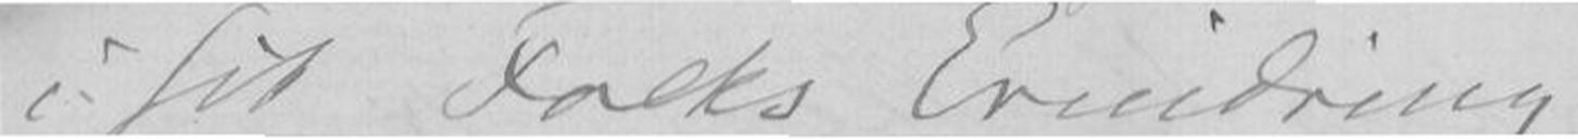i sit Folks Erindring,
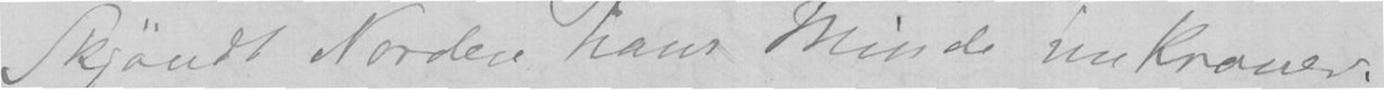Skjøndt Norden hans Minde nu Kroner.
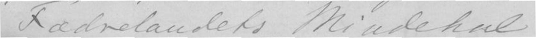Fædrelandets Mindretal
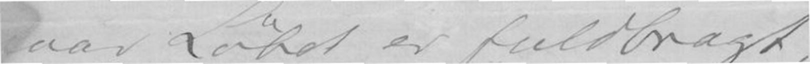var Løbet er fuldbragt,
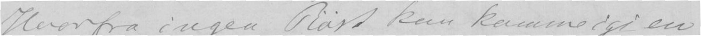Hvorfra ingen Røst kan komme igjen
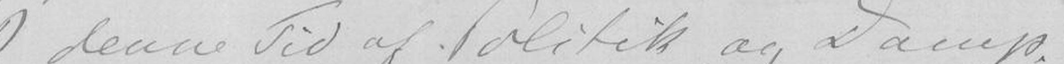I denne Tid af Politik og Damp.
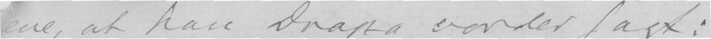ene, at han Drapa vorder sagt:
